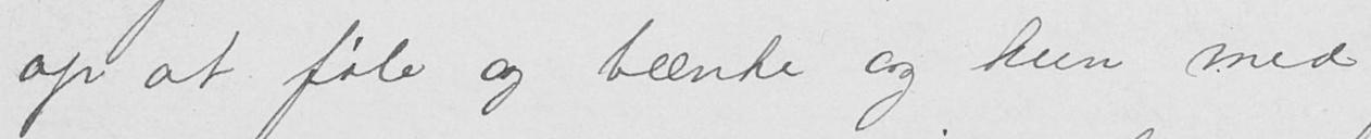op at føle og tænke og kun med
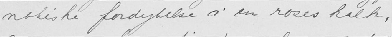notiske fordybelse i en roses kalk,
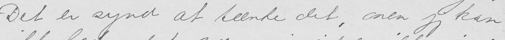Det er synd at tænke det, men jeg kan
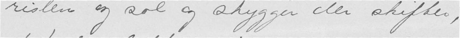rislen og sol og skygger der skifter,
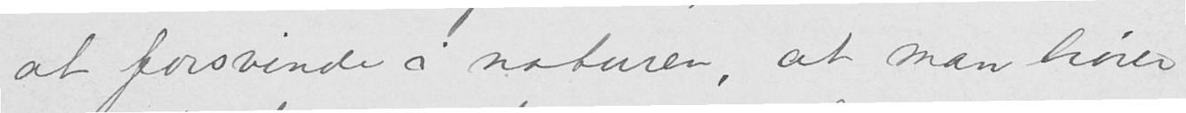at forsvinde i naturen, at man hører
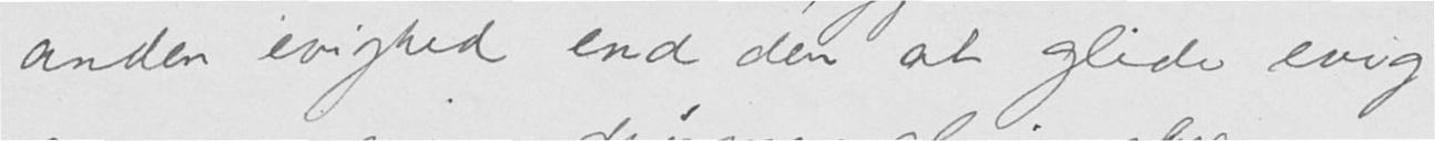anden evighed end den at glide evig
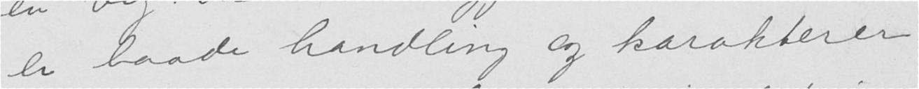er baade handling og karakterer
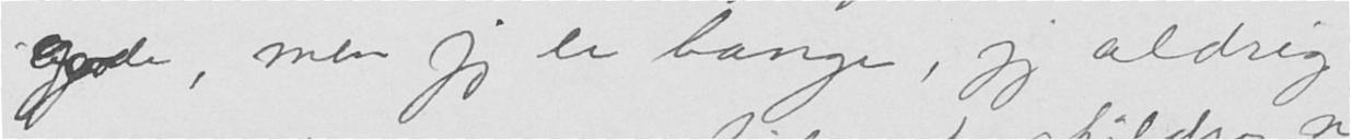gode, men jeg er bange, jeg aldrig
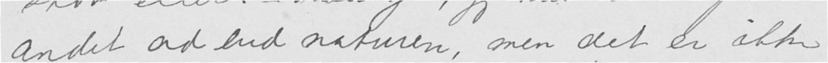andet ord end naturen, men det er ikke
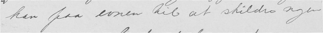kan faa evnen til at skildre nogen
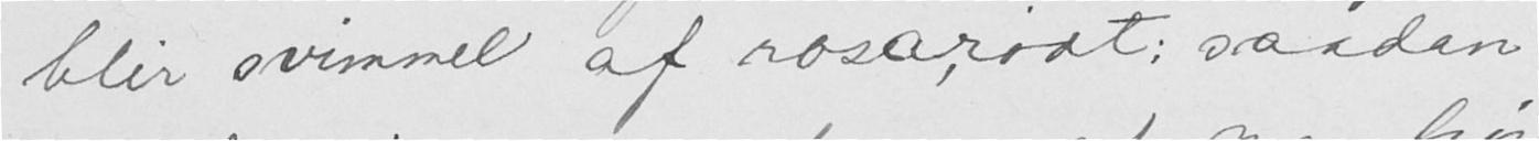blir svimmel af rosarødt: saadan
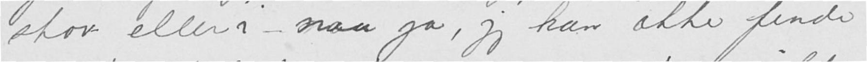skov eller i - naa ja, jeg kan ikke finde
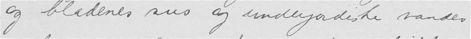og bladenes sus og underjordiske vander
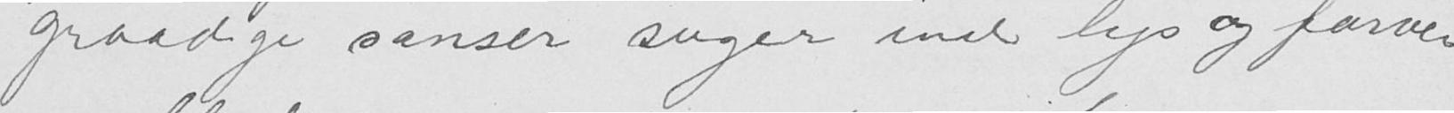graadige sanser suger ind lys og farver
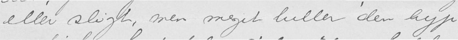eller sligt, men meget heller den hyp-
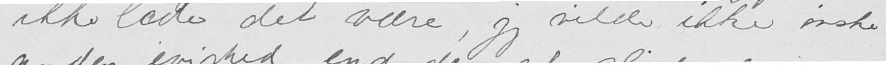ikke lade det være, jeg vilde ikke ønske
